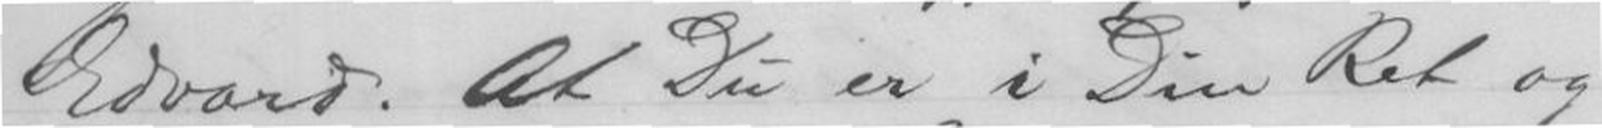Edvard. At Du er i Din Ret og
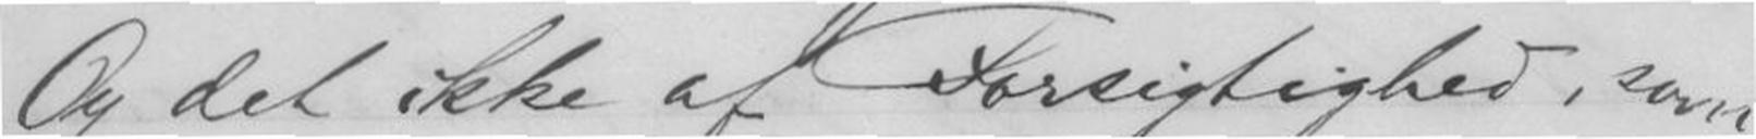Og det ikke af Forsigtighed, som
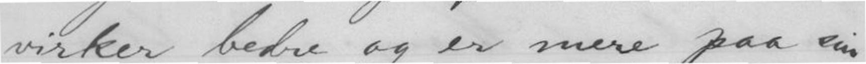virker bedre og er mere paa sin
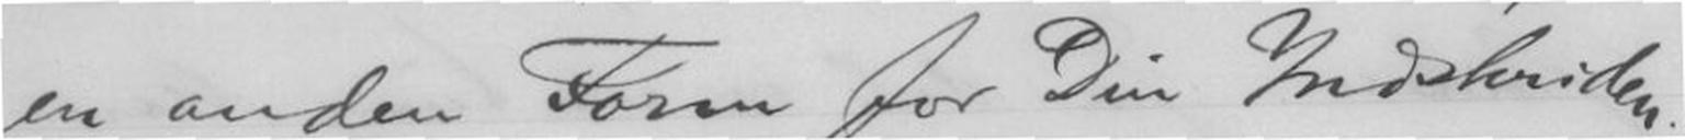en anden Form for Din Indskriden.
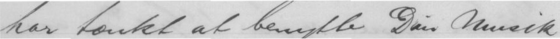har tænkt at benytte Din Musik
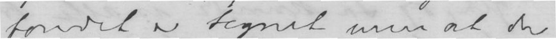fondet er tegnet men at der
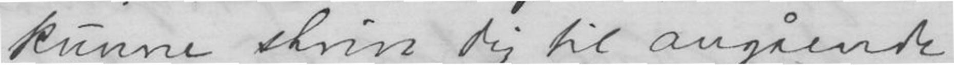kunne skrive dig til angående
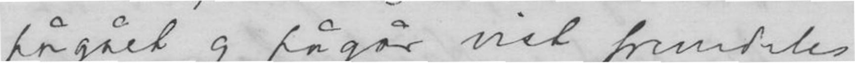pågået og pågår vist fremdeles
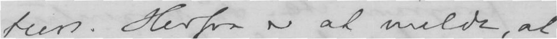teen. Herfra at melde, at
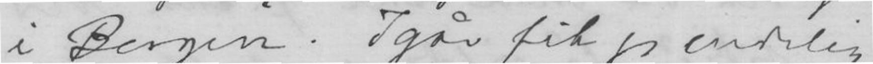i Bergen. Igår fik jeg endelig
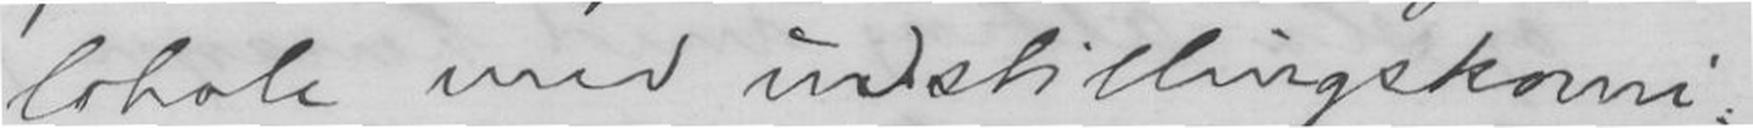lokale med instillingskomi-
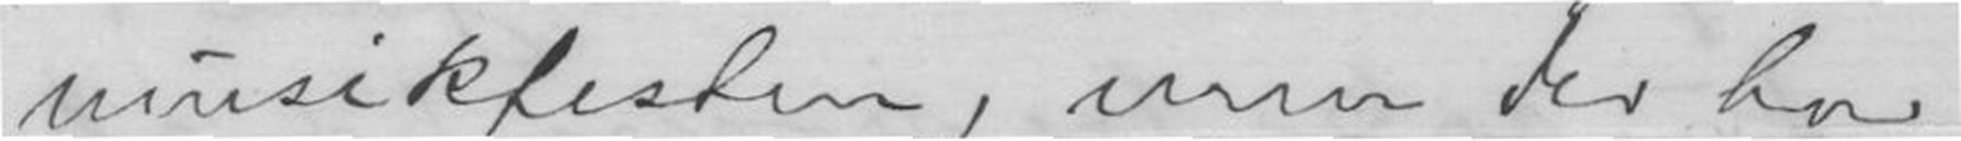musikfesten, men der har
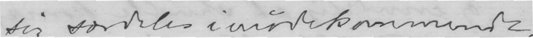sig særdeles imødekommende,
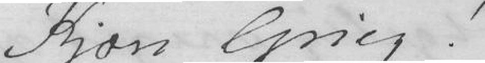Kjære Grieg!
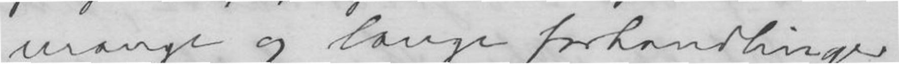mange og lange forhandlinger
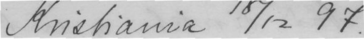Kristiania 18/12 97
